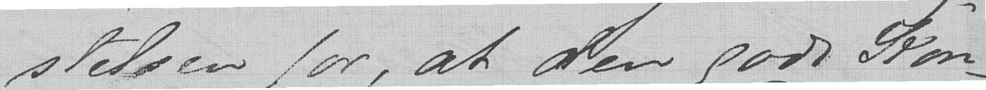stelsen for, at den gode Kon-
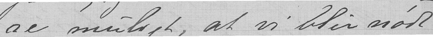re muligt, at vi blir nødt
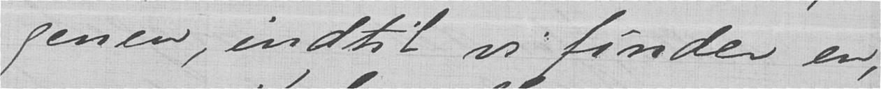genen, indtil vi finder en
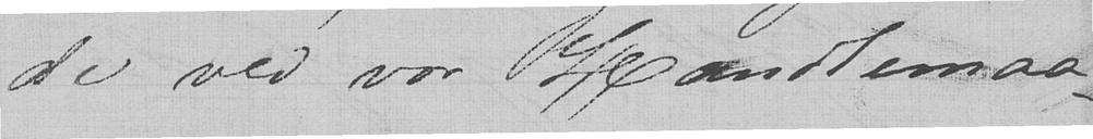de ved vor Handlemaa-
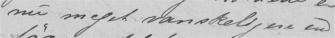nu meget vanskeligere end
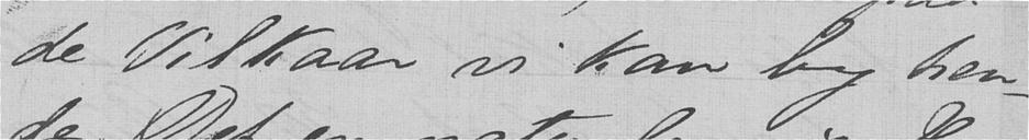de Vilkaar vi kan by hen-
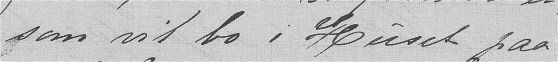som vil bo i Huset paa
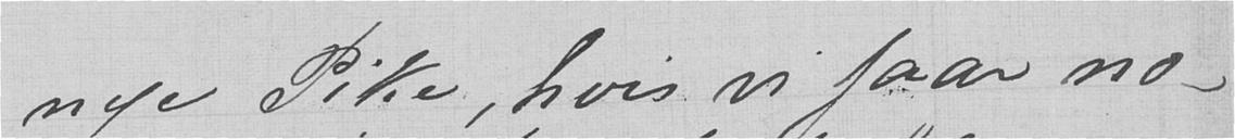nye Pike, hvis vi faar no-
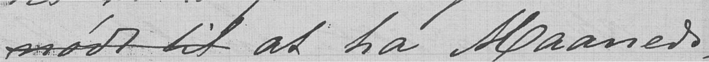nødt til at ha Maaneds-
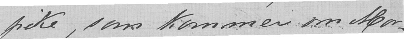pike, som kommer om Mor-
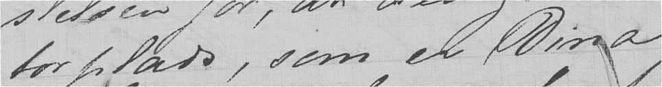torplads, som er Dina
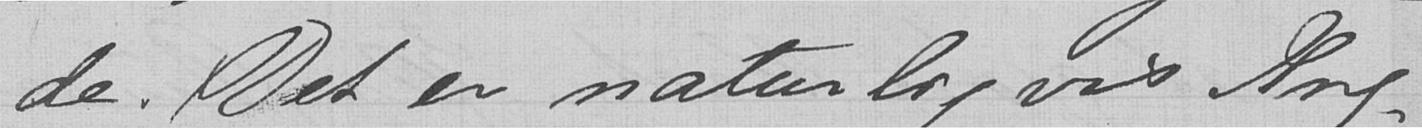de. Det er naturligvis Æng-
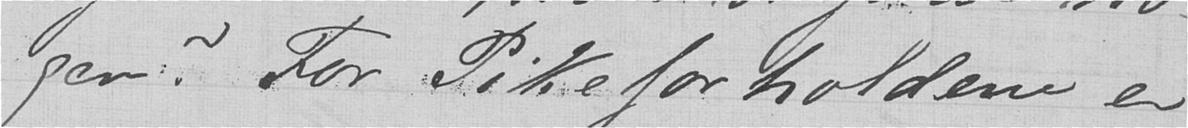gen? For Pikeforholdene er
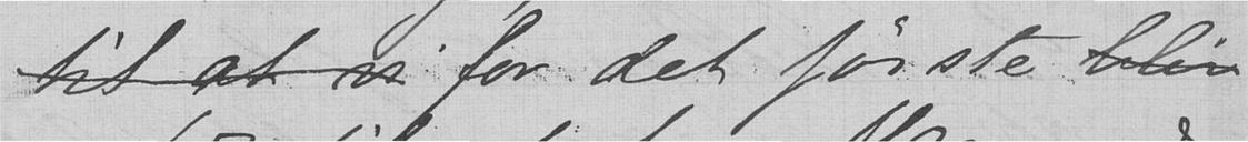til at vi for det første blir
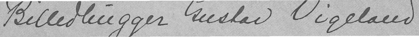Billedhuggeren Gustav Vigeland
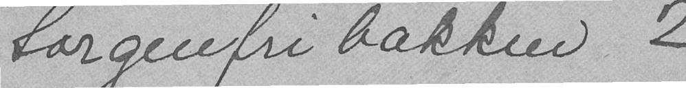Sorgenfribakken 2
